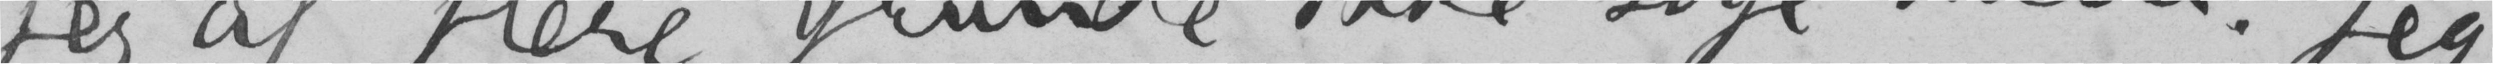jeg af flere grunde ikke søge ham. Jeg
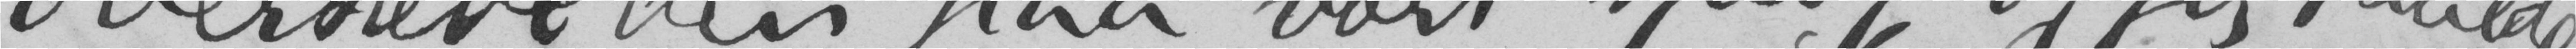oversætte den paa vort sprog, og jeg skulde
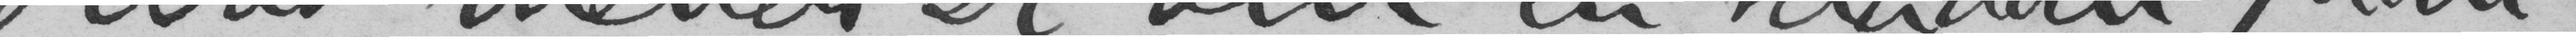Hvad mener De om en saadan plan?
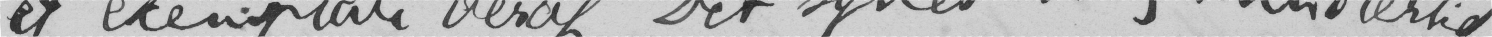et exemplar deraf. Det synes mig imidlertid
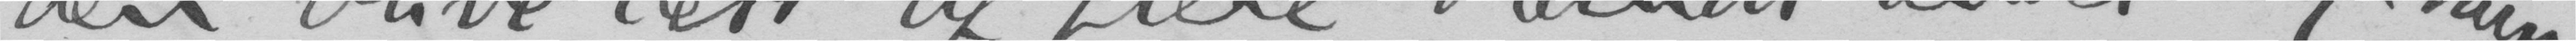den blive læst af flere, blant andet af min
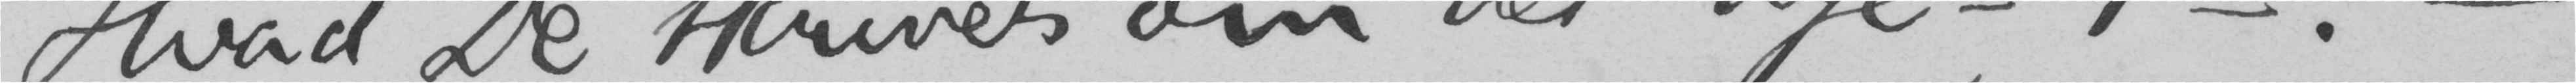Hvad de skriver om det nye - 7de! -
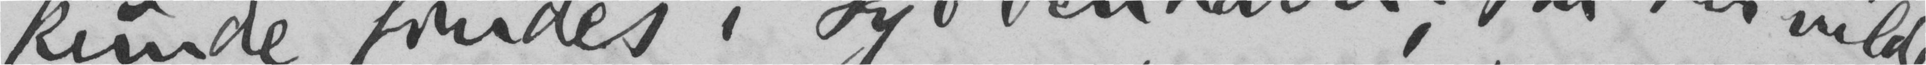kunde findes i Kjøbenhavn; thi her vilde
